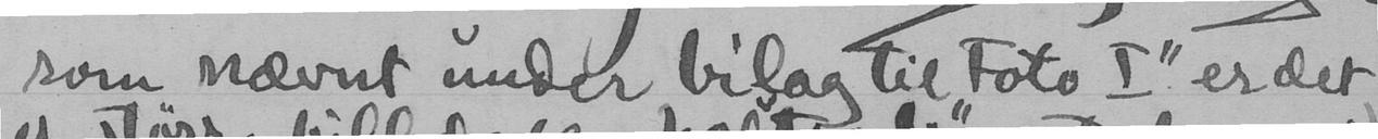som nævnt under bilag til "Foto I" er det
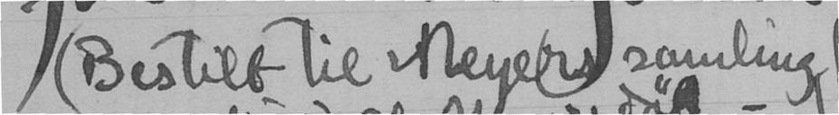(Bestilt til Meyers samling
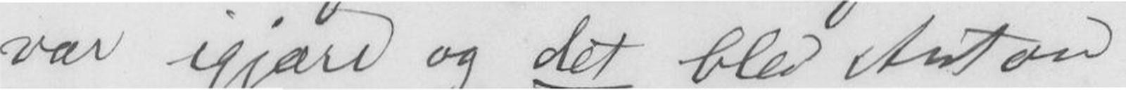var igjære og det blev Anton
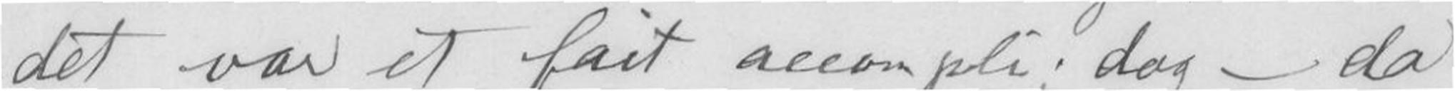det var et fait accompli; dog - da
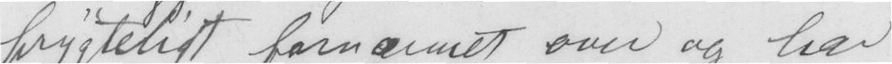frygteligt fornærmet over og har
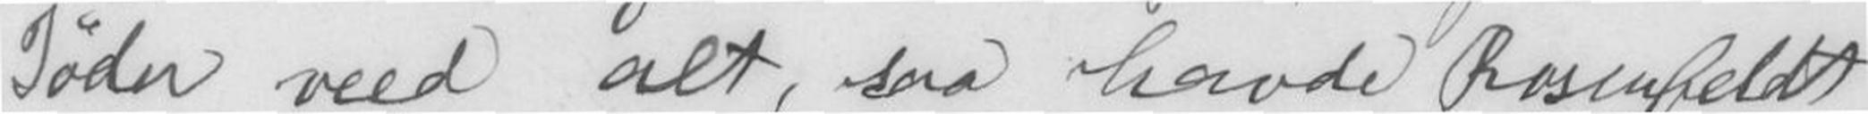Jøder veed alt, saa havde Rosenfeldt
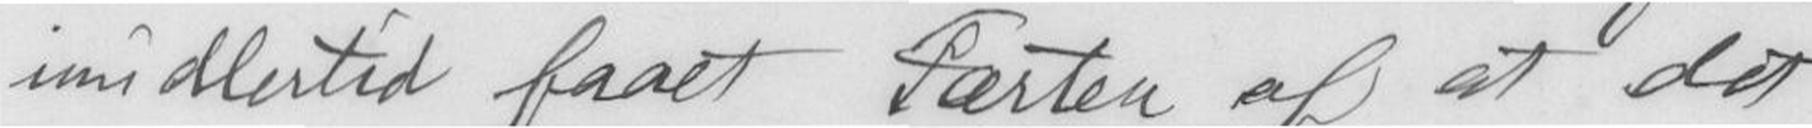imidlertid faaet Færten af at det
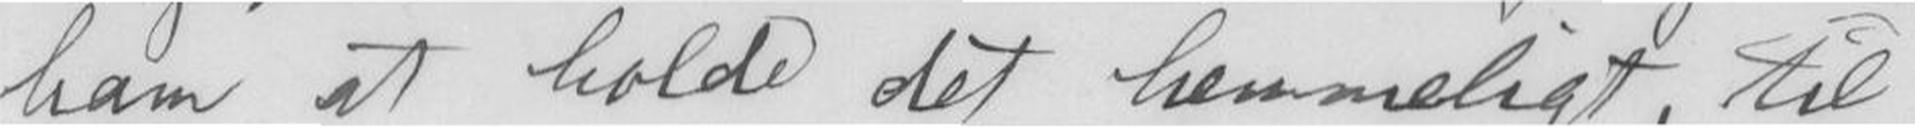ham at holde det hemmeligt, til
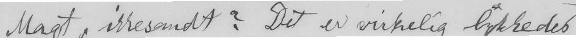Magt, ikkesandt? Det er virkelig lykkedes
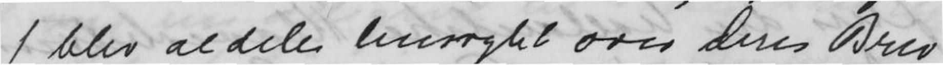J blev aldeles henrykt over Deres Brev
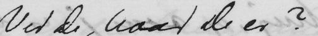Ved De, hvad De er?
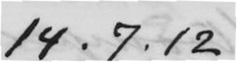14.7.12
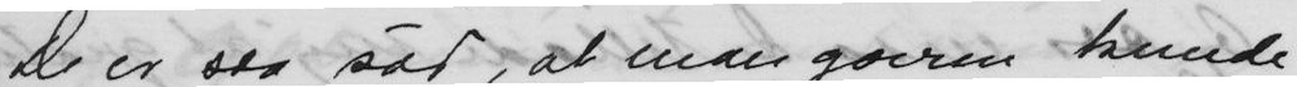De er saa sød, at man gaerne kunde
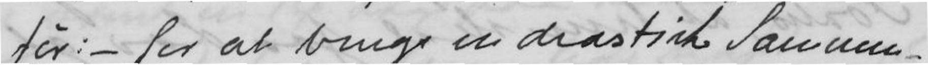før. - for at bruge en drastisk Sammen-
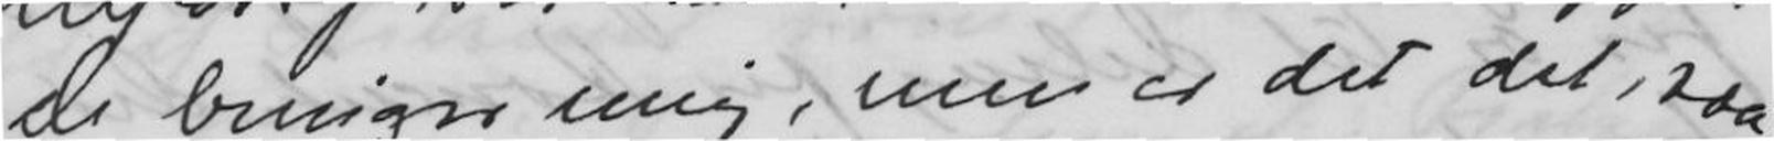De bringer mig, men er det det, saa
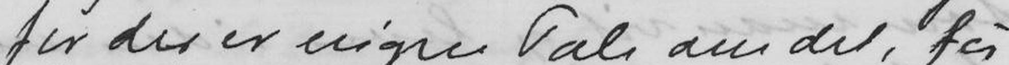for der er ingen Tale om det, før
